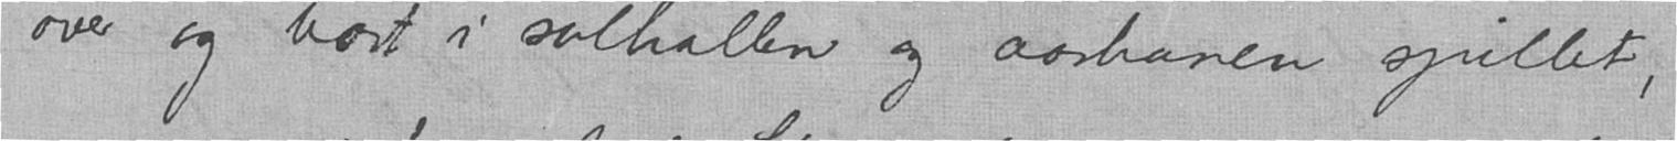over og bart i solhallen og aarhanen spillet,
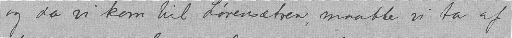og da vi kom til Lørensætren, maatte vi ta af
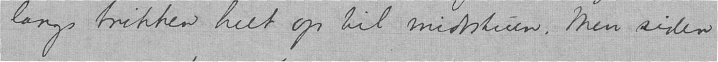langs trikken helt op til midtstuen. Men siden
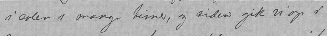i solen i mange timer, og siden gik vi op i
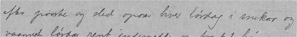efter paaske og sled opover hver lørdag i snekav og
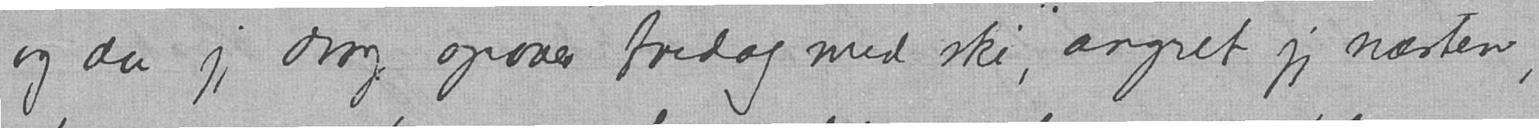og da jeg drog opover fredag med ski angret jeg næsten,
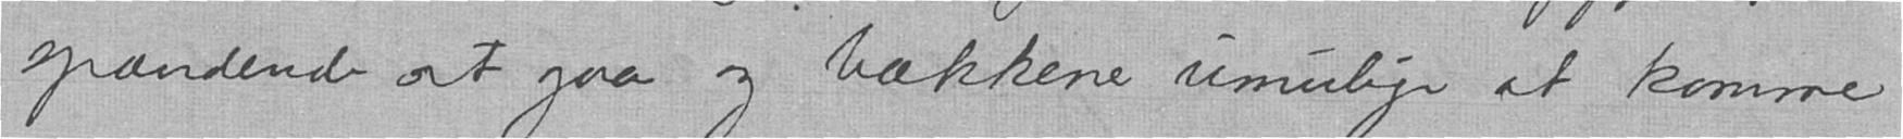spændende at gaa og bækkene umulige at komme
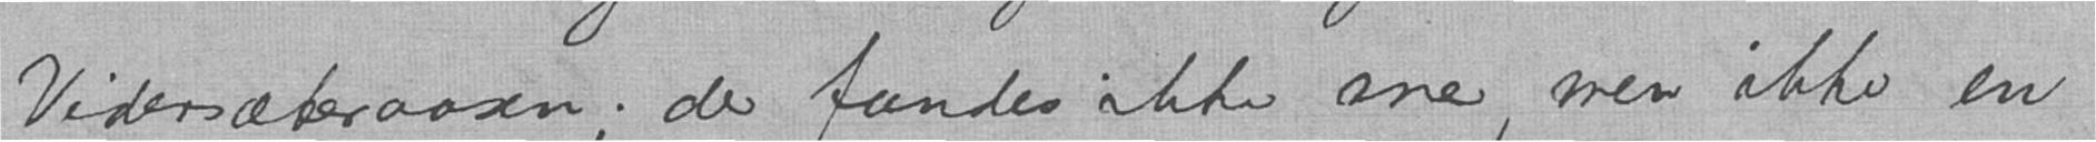Vidersæteraasen; der fandes ikke sne, men ikke en
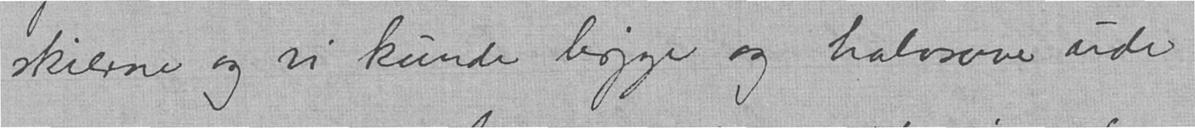skierne og vi kunde ligge og halvsove ude
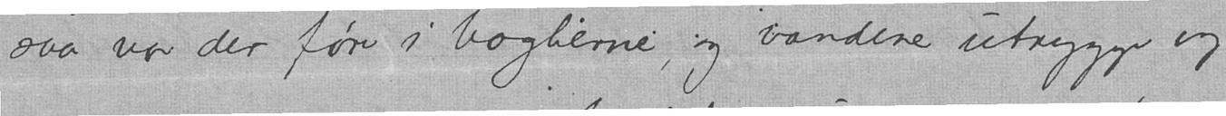saa var der føre i baglierne, og vandene utrygge og
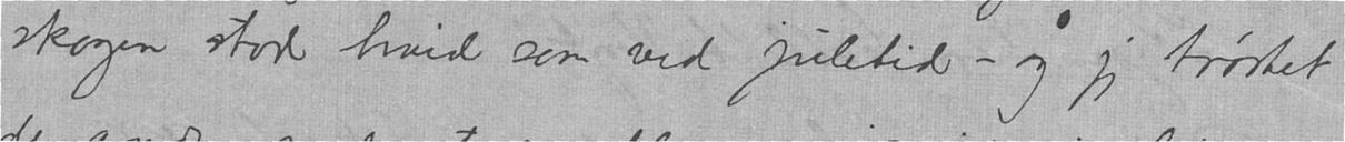skogen stod hvid som ved juletid - og jeg trøstet
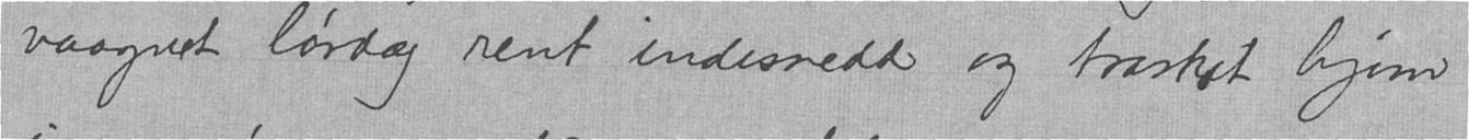vaagnet lørdag rent indesnedd og trasket hjem
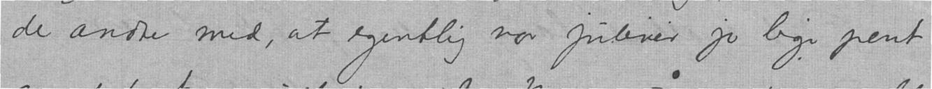de andre med, at egentlig var juleveir jo lige pent
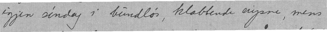igjen søndag i bundløs, klabbende nysne, mens
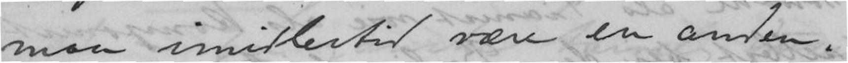maa imidlertid være en anden.
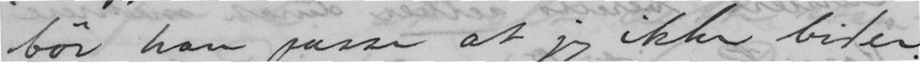bör han passe at jeg ikke bider.
